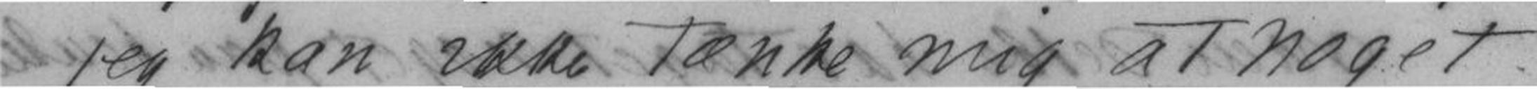jeg kan ikke tænke mig at noget
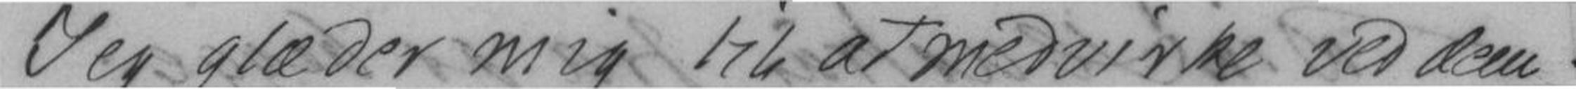Jeg glæder mig til at medvirke ved dem.
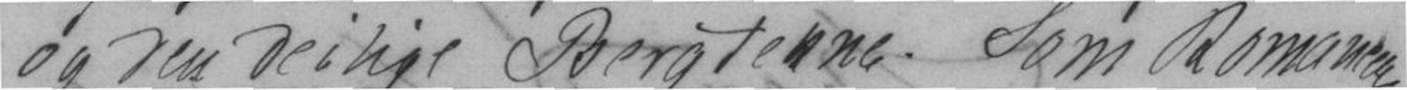og den deilige Bergtekne. Som Romancen
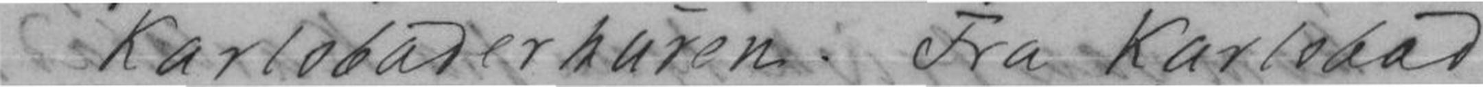Karlstader turen. Fra Karlstad
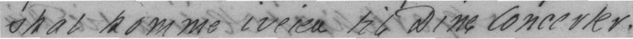skal komme iveien til Dine Concerter.
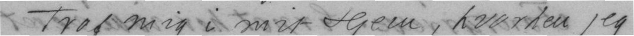Traf mig i mit Hjem, hvorhen jeg
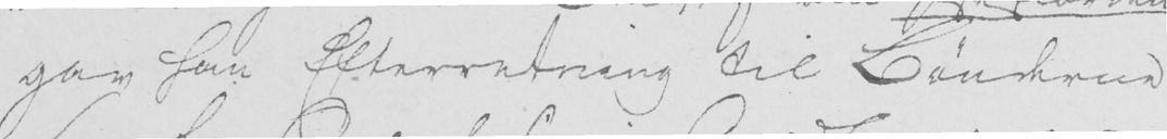gav han efterretning til Bønderne
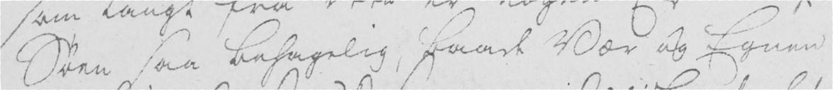Søen saa behagelig, baade Vær og Egnen
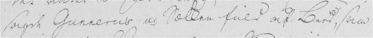sagde Gunnerus og Sækken fuld af Brød, saa
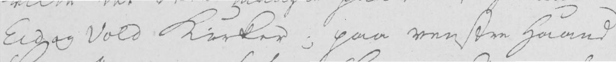Eid og Vold Kirker; paa venstre Haand
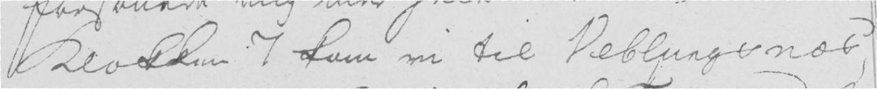Klokken 7 kom vi til Veblungsnæs,
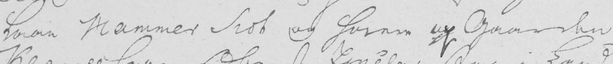Laae Hammer Skot og høiere  Gaarden
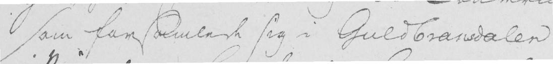som forsamlede sig i Guldbransdalen
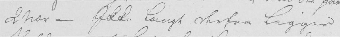2 nær - Ikke langt derfra ligger
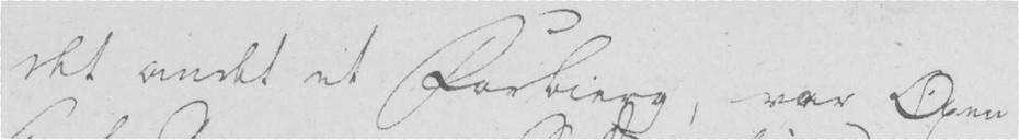det andet et Forbierg, var Oxen
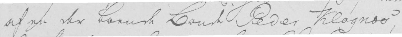af en der boende Bonde Peder Klognæs,
